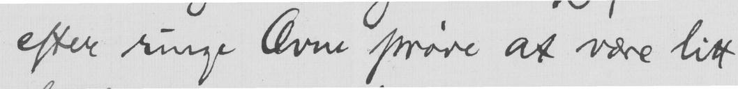efter ringe Ævne prøve at være litt
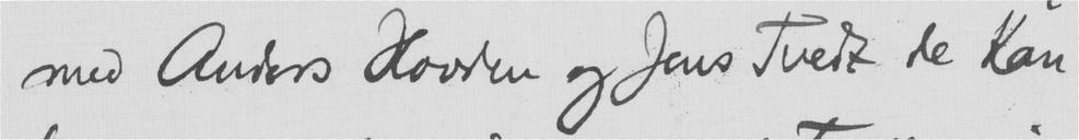med Anders Hovden og Jens Tvedt de kan
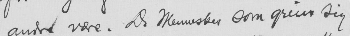andre være. De Mennesker som greier sig
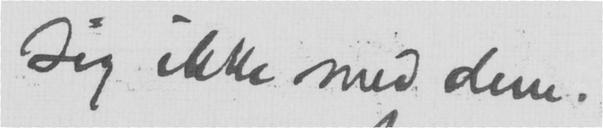sig ikke med dem.
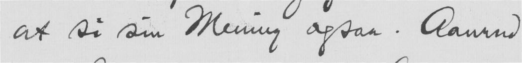at si sin Mening ogsaa. Aanrud
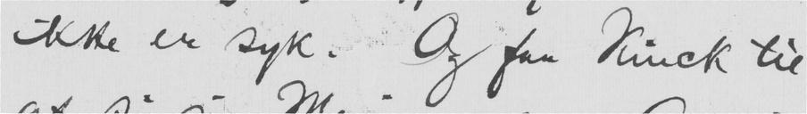ikke er syk. Og faa Kinck til
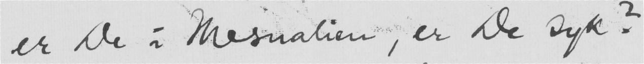er De i Mesnalien, er De syk?
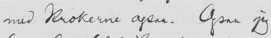med Krokerne ogsaa. Ogsaa jeg
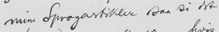mine Sprogartikler saa si det
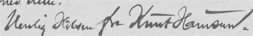Venlig Hilsen fra Knut Hamsun.
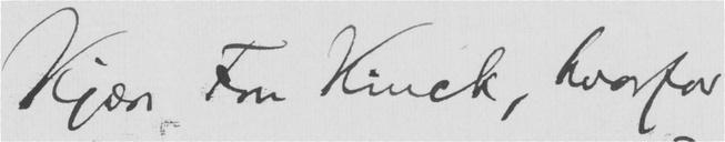Kjære Fru Kinck, hvorfor
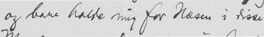og bare holde mig for Næsen i disse
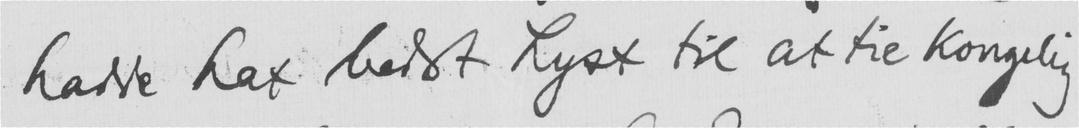hadde hat bedst Lyst til at tie kongelig
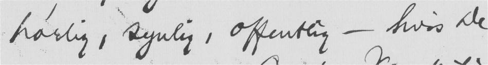hørlig, synlig, offentlig - hvis De
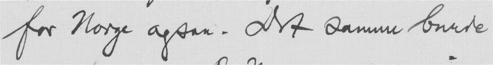for Norge ogsaa. Det samme burde
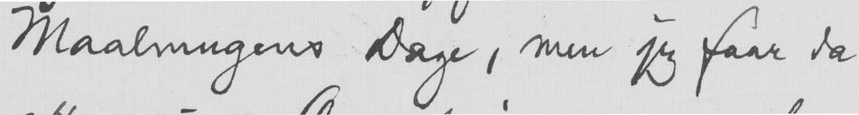Maalmugens Dage, men jeg faar da
